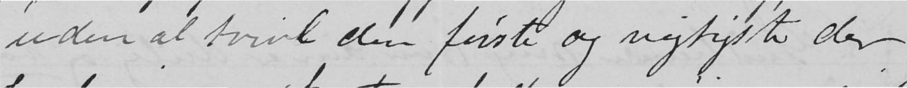uden al tvivl den første og vigtigste der
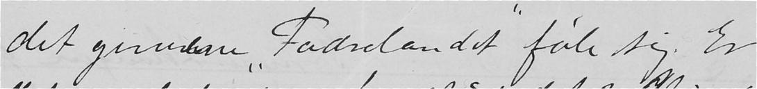det givndee "Fædrelandet" føle sig. Er
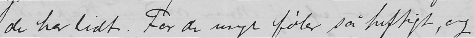de har lidt. For de unge føler så heftigt, og
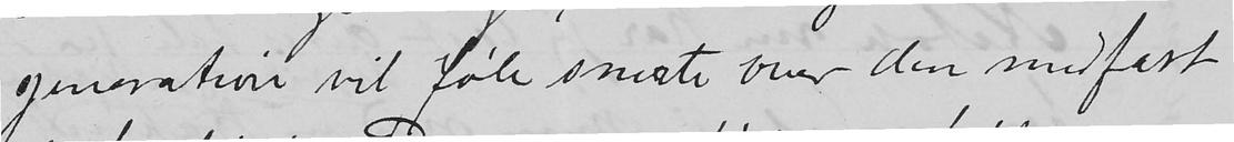generation vil føle smærte over den medfart
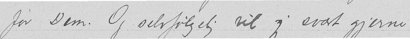for Dem. Og selvfølgelig vil jeg svært gjerne
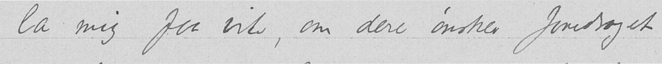la mig faa vite, om dere ønsker foredraget
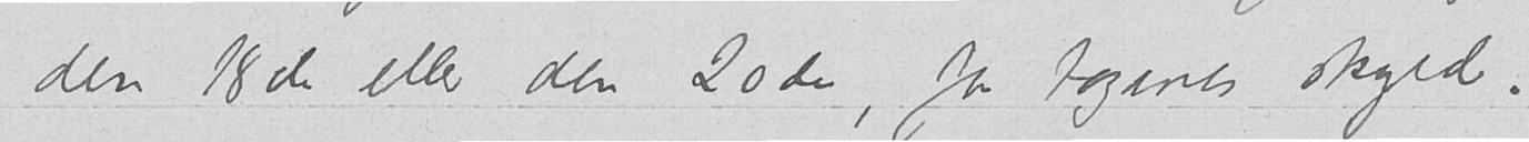den 18de eller den 20de, for togenes skyld.
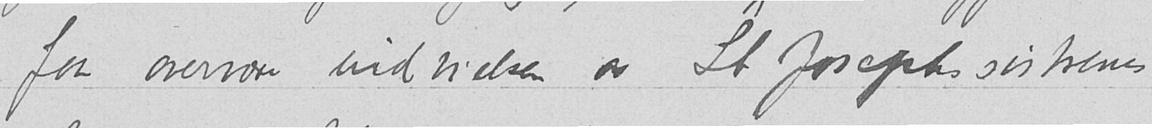faa overvære indvielsen av St. Josephssøstrenes
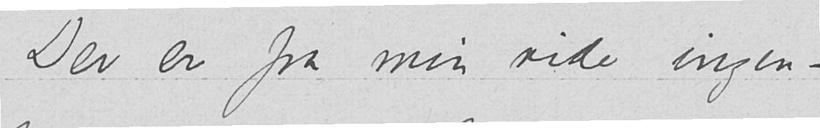Der er fra min side ingen-
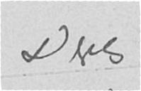Deres
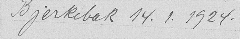Bjerkebæk 14.1.1924.
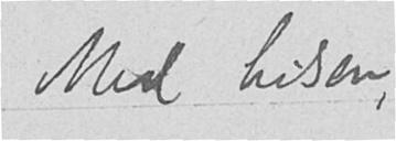Med hilsen,
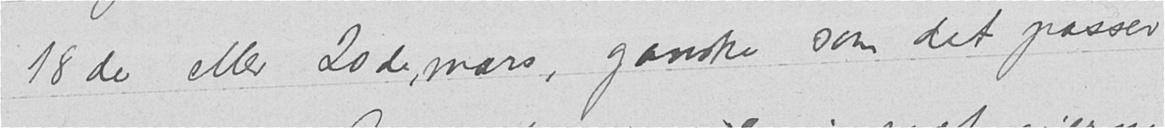18de eller 20de, mars, ganske som det passer
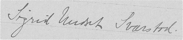Sigrid Undset Svarstad.
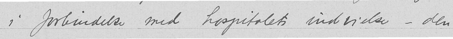i forbindelse med hospitalets indvielse – den
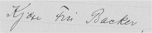Kjære Fru Backer,
